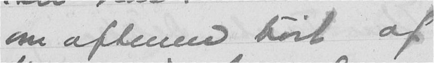om aftenen høit af
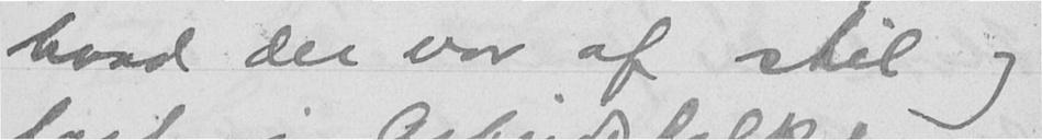hvad der var af stil og
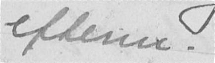efterm.
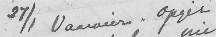27/1 Vaarveir. opgir
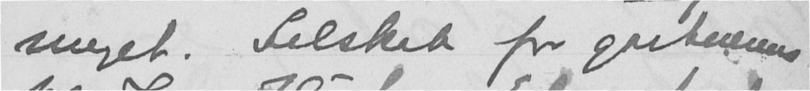meget. Selskab for gartnerens
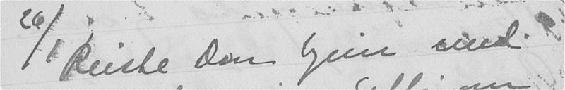26/1 Reiste Don hjem med
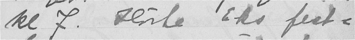kl 7. Hørte Eks fest-
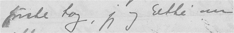første tog, jeg og Etti om
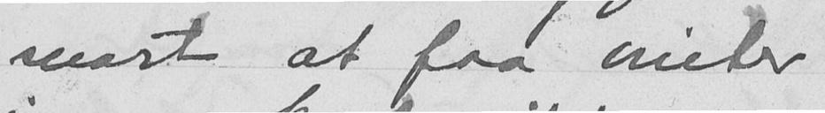snart at faa vinter
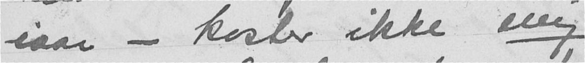iaar - koster ikke mig
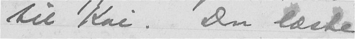til Kai. Don læste
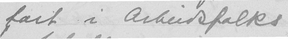fart i Arbeidsfolks
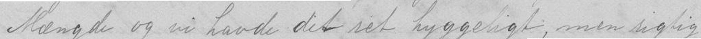Mængde og vi havde det ret hyggeligt, men rigtig
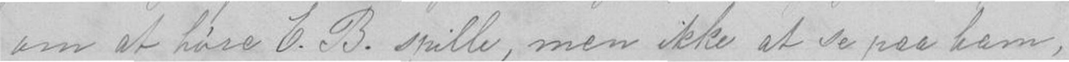om at høre E.B. spille, men ikke at se paa ham,
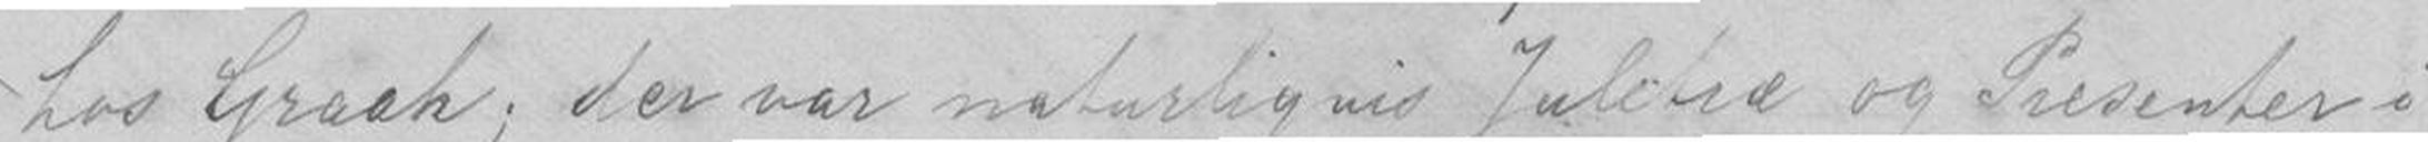hos Graah; der var naturligvis Juletræ og Presenter i
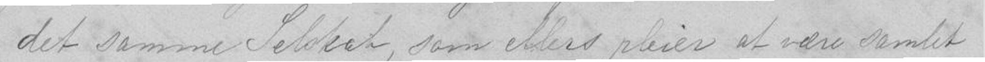det samme Selskab, som ellers pleier at være samlet
